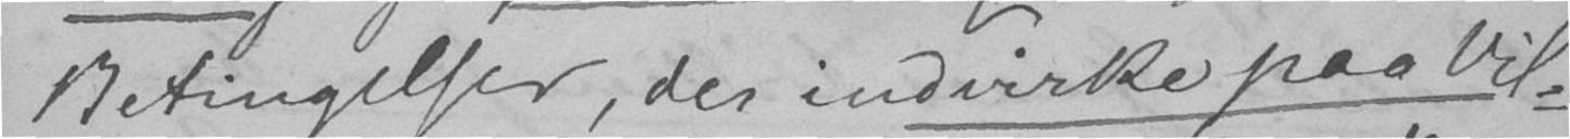Betingelser, der indvirke paa Vil-
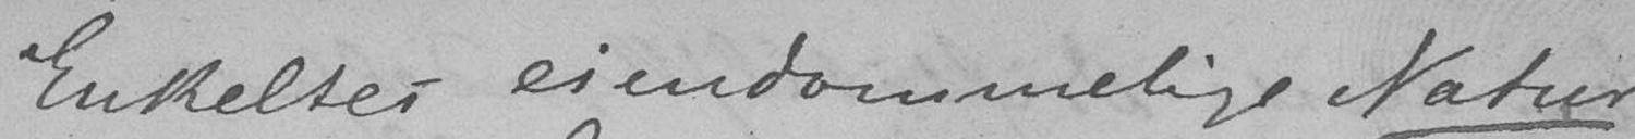Enkeltes eiendommelige Natur-
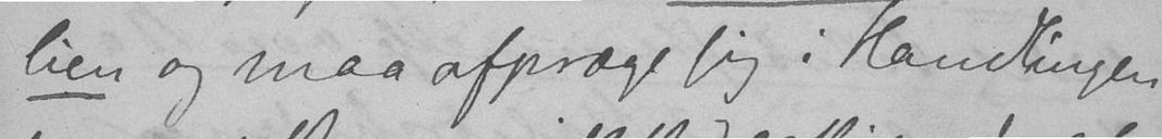lien og maa afpræge sig i Handlingen
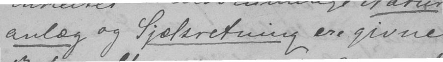anlæg og Sjælsretning ere givne
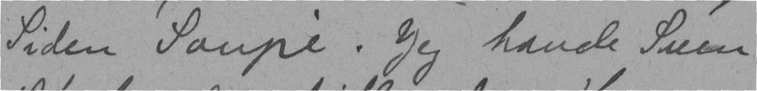Siden Soupè. Jeg havde Sven
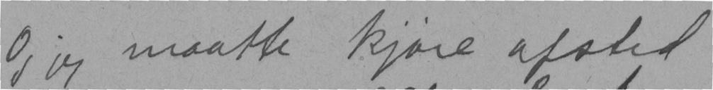Og jeg maatte kjøre afsted
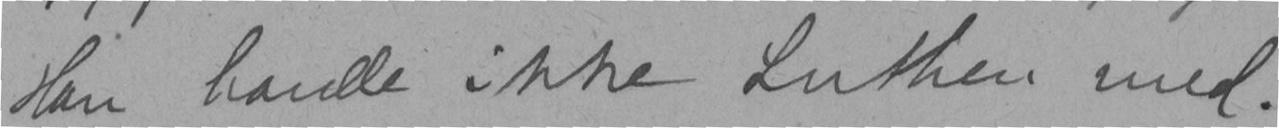Han havde ikke Luthen med.
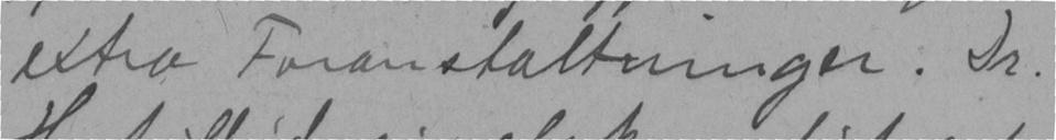extra Foranstaltninger. Dr.
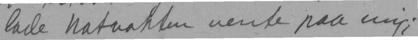lade Natvakten vente paa mig;
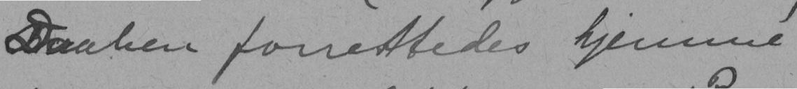Daaben forrettedes hjemme
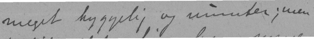meget hyggelig og munter; men
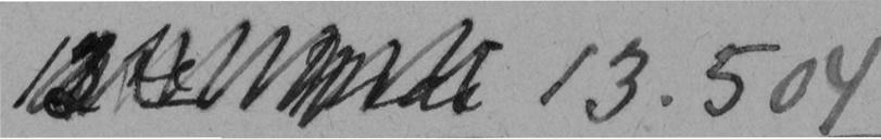13.5 04
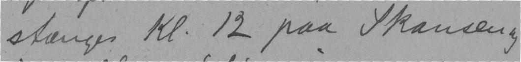stænges Kl. 12 paa Skansen og
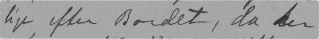lige efter Bordet, da der
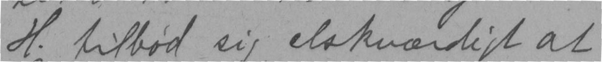H. tilbød sig elskværdigt at
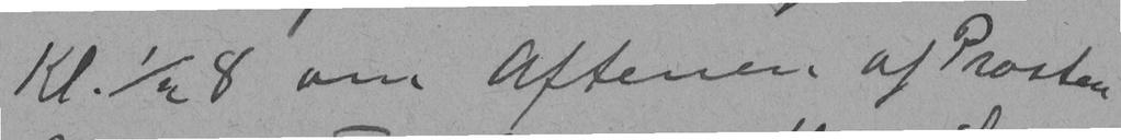Kl. 1/2 8 om Aftenen af Prosten
